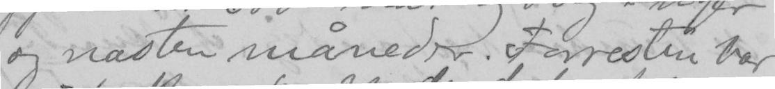og næsten måneder. Forresten var
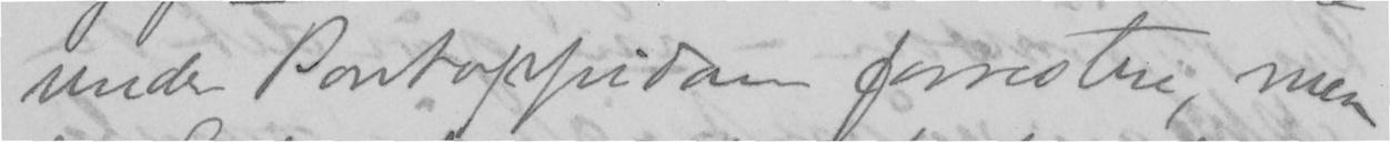under Pontoppidan forresten, men
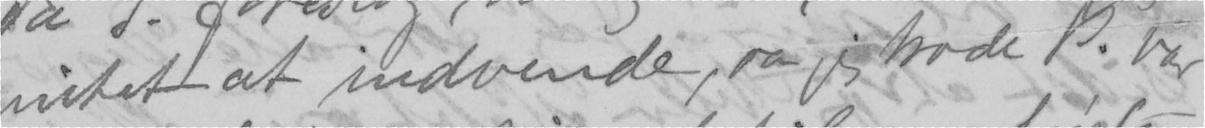intet at indvende, da jeg trode P. var
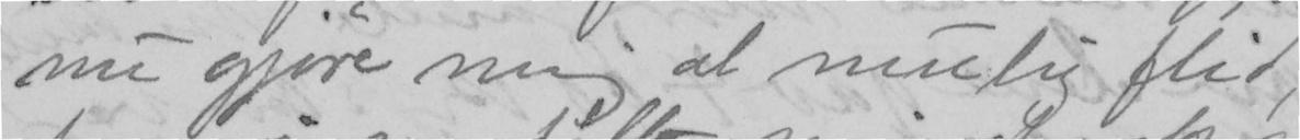nu gjøre mig al mulig flid,
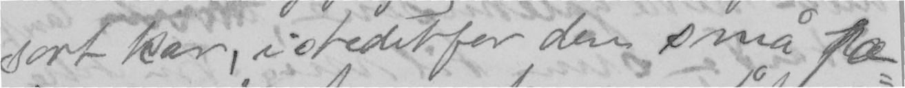sort kar, i stedetfor den små pæ-
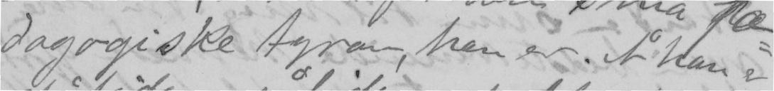dagogiske tyran, han er. Å han er
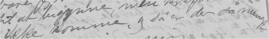ikke komme, og så er der så mange
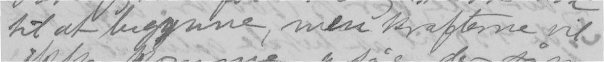til at begynne, men kræfterne vil
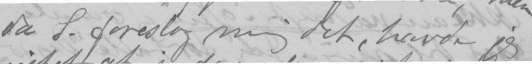da S. foreslog mig det, havde jeg
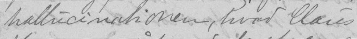hallucinationen, hvad Claus
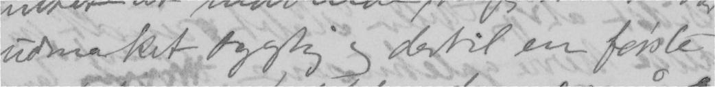udmærket dygtig og dertil en første-
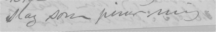slag som piner mig.
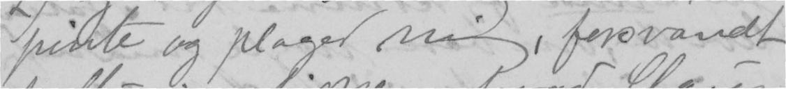pinte og plaged mig, forsvandt
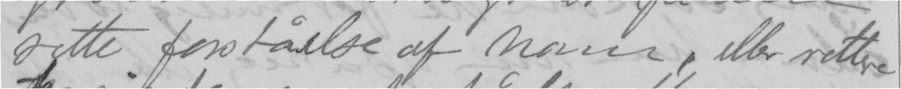rette forståelse af ham, eller rettere
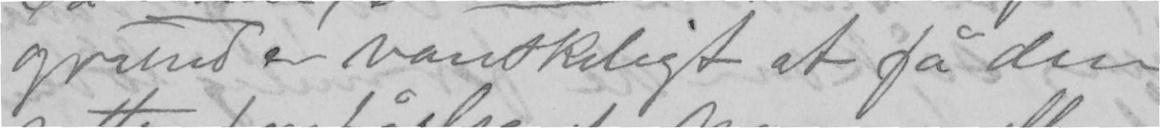grund er vanskeligt at få den
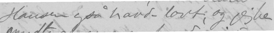Hansen også havde lovt, og jeg be
